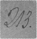213.
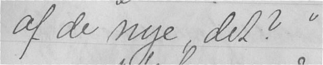af de nye, det?"
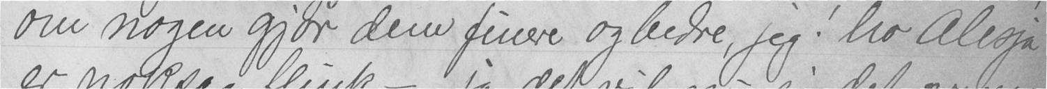om nogen gjør dem finere og bedre, jeg! ho Alesja
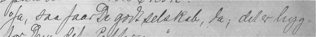"Ja, saa faar De godt selskab, da; det er hyg-
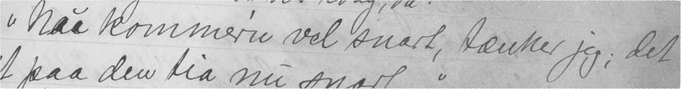"Naa kommer'n vel snart, tænker jeg; det
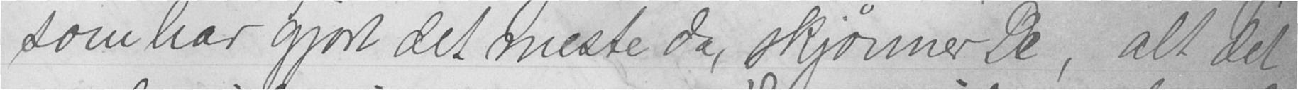som har gjort det meste da, skjønner De, alt det
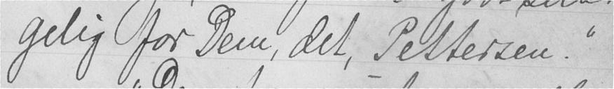gelig for Dem, det, Pettersen. "
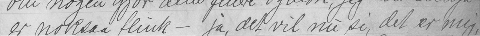er noksaa flink - ja, det vil nu si, det er mig,
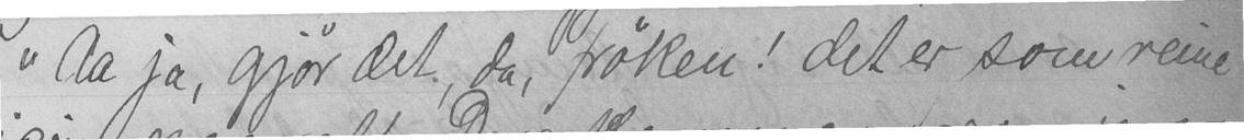"Aa ja, gjør det, da, frøken! det er som reine
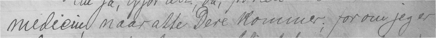medicin, naar atte Dere kommer; for om jeg er
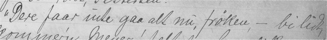"Dere faar inte gaa alt nu, frøken, - bi lidt,
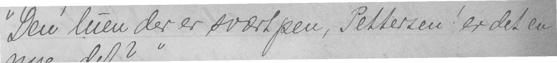"Den luen der er svært pen, Pettersen! er det en
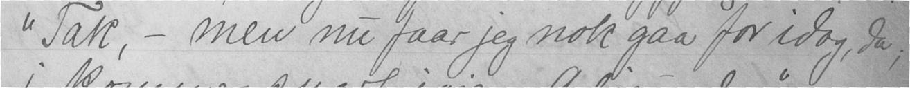"Tak, - men nu faar jeg nok gaa for idag, da;
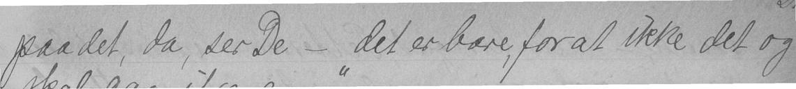paa det, da, ser De - det er bare, for at ikke det og
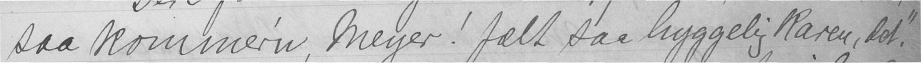saa kommer'n, Meyer! fælt saa hyggelig karen, det."
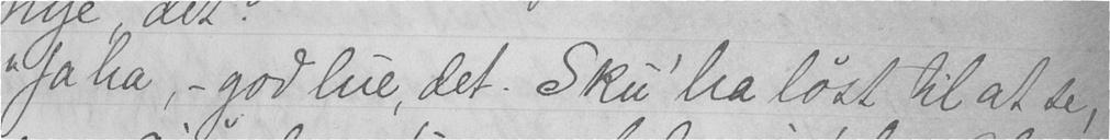"Ja ha, - god lue, det. Sku' ha løst til at se,
